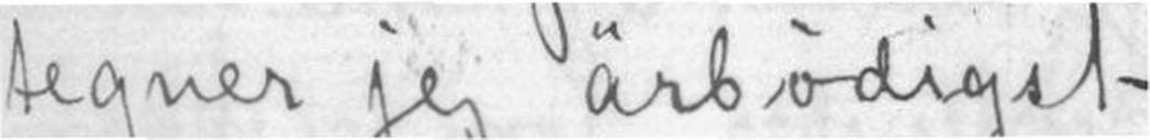tegner jeg ärbødigst -
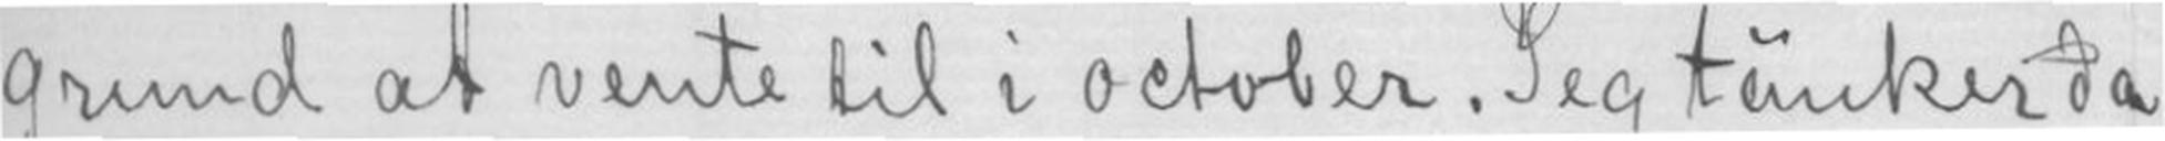grund at vente til i october. Jeg tänker da
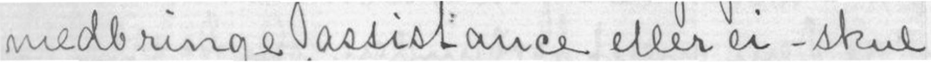medbringe assistanse eller ei - skul-
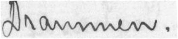Drammen.
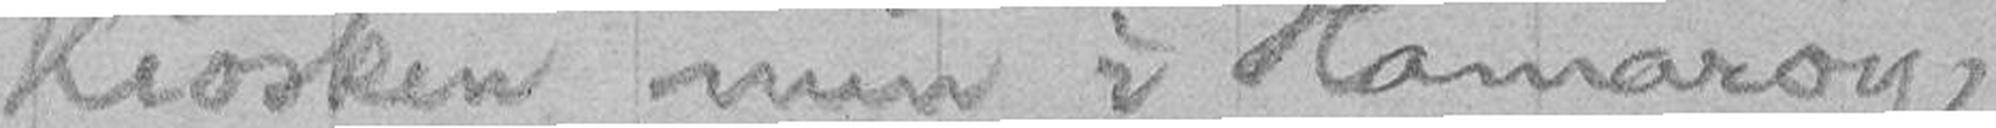Kiosken min i Hamarøy
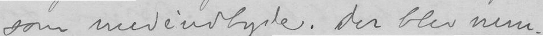som medindbyder. Der blev nem-
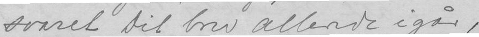svaret Dit brev allerede igår,
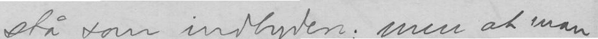stå som indbytere; men at man
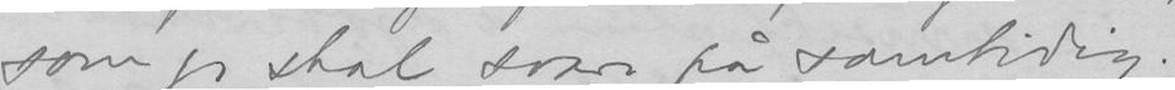som jeg skal svare på samtidig.
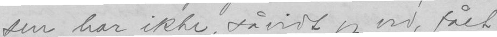sen har ikke, såvidt jeg ved, fået
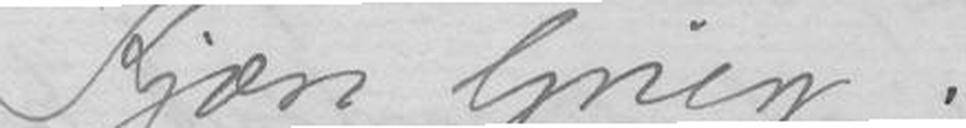Kjære Grieg!
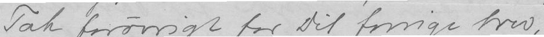Tak forøvrigt for Dit forrige brev,
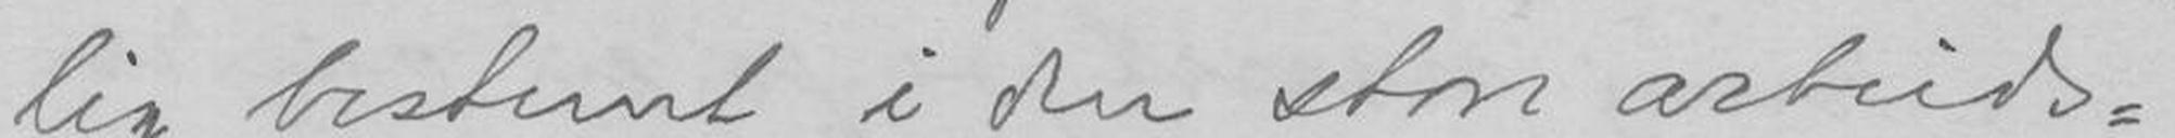lig bestemt i den store arbeids-
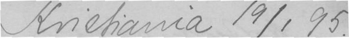Kristiania 19/1 95
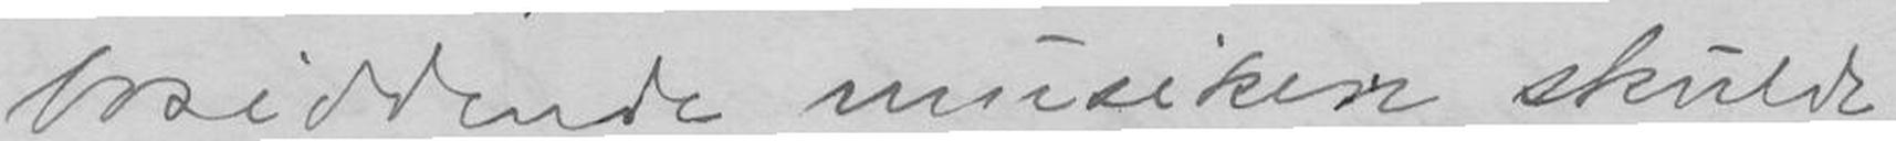bosiddende musikere skulde
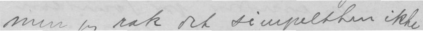men jeg rak det simpelthen ikke.
